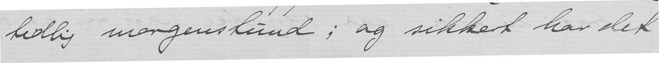tidlig morgenstund; og sikkert har det
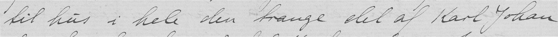til hus i hele den trange del af Karl Johan
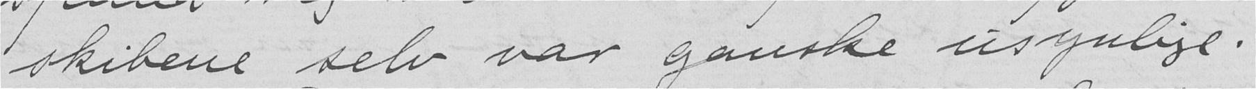skibene selv var ganske usynlige.
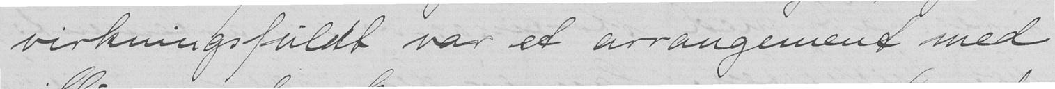virkningsfuldt var et arrangement med
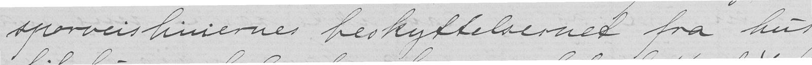sporveisliniernes beskyttelsesnet fra hus
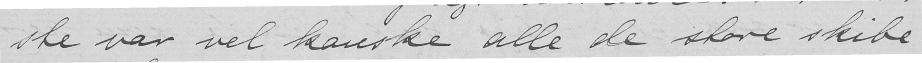ste var vel kanske alle de store skibe
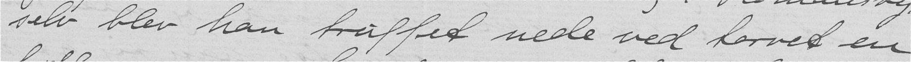selv blev han truffet nede ved torvet en
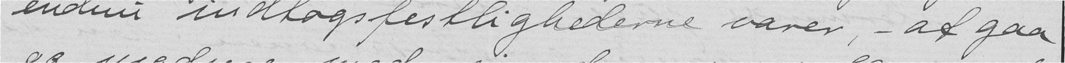endnu indtogsfestlighederne varer, – at gaa
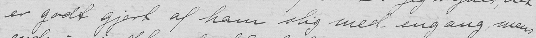er godt gjort af ham slig med engang, mens
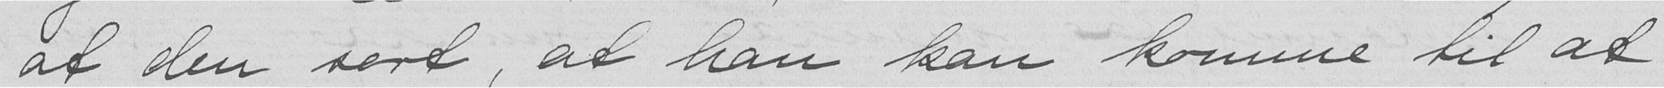af den sort, at han kan komme til at
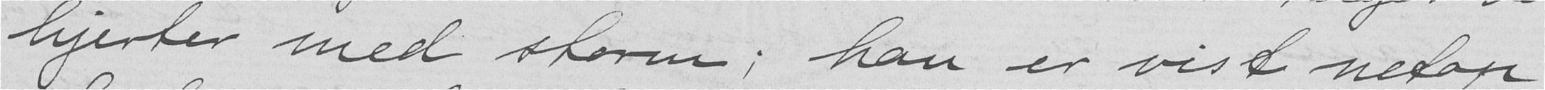hjerter med storm; han er vist netop
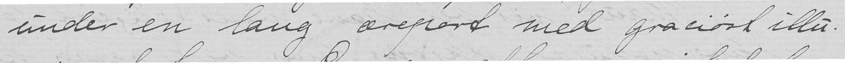under en lang æreport med graciøst illu-
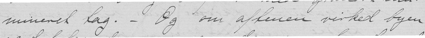mineret tag. – Og om aftenen virked byen
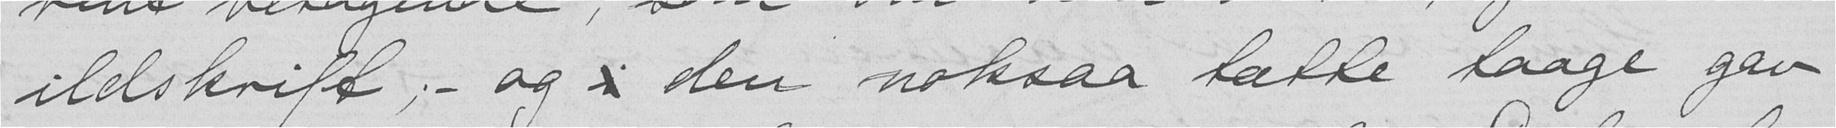ildskrift; – og i den noksaa tætte taage gav
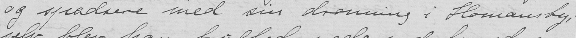og spadsere med sin dronning i Homansby;
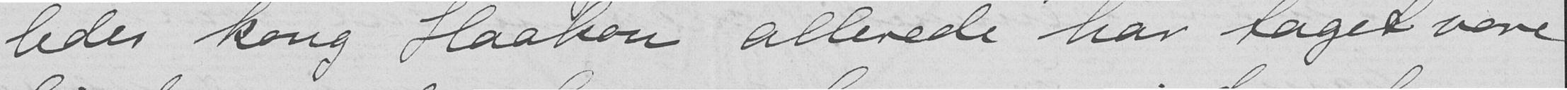ledes kong Haakon allerede har taget vore
